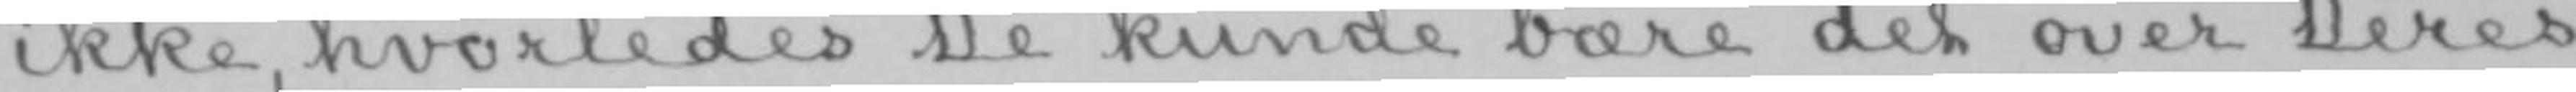ikke, hvorledes De kunde bære det over Deres
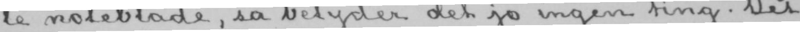le noteblade, så betyder det jo ingen ting. Det
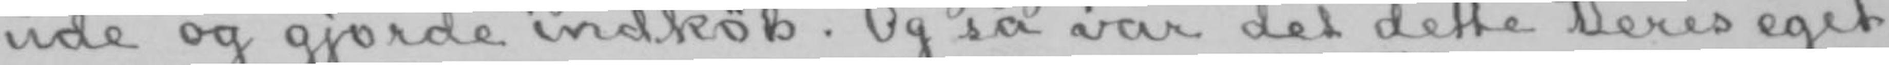ude og gjorde indkøb. Og så var det dette Deres eget
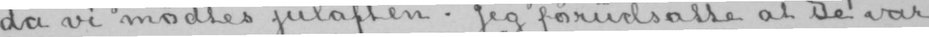da vi mødtes julaften. Jeg forudsatte at De var
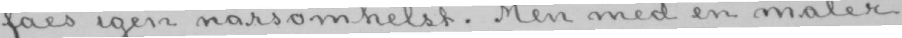fåes igen nårsomhelst. Men med en maler
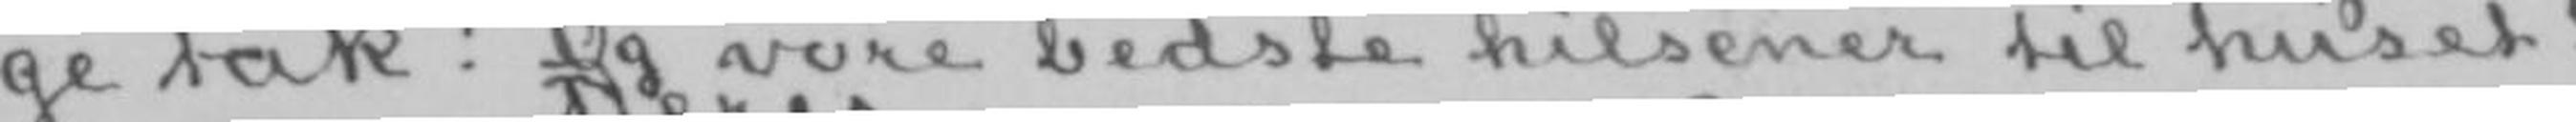ge tak! Og vore bedste hilsener til huset!
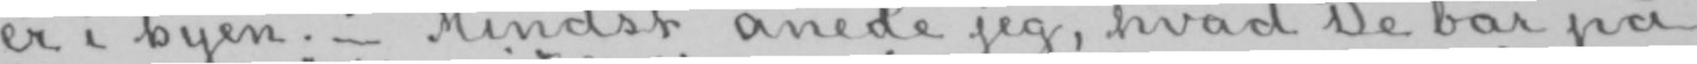er i byen.- Mindst anede jeg, hvad De bar på
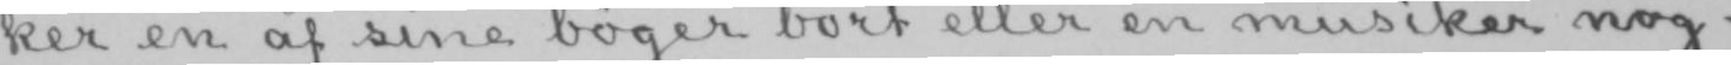ker en af sine bøger bort eller en musiker nog-
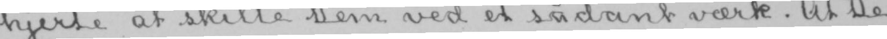hjerte at skille Dem ved et sådant værk. At De
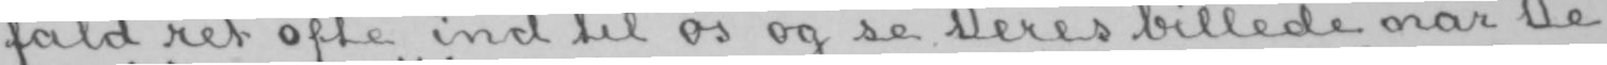fald ret ofte ind til os og se Deres billede når De
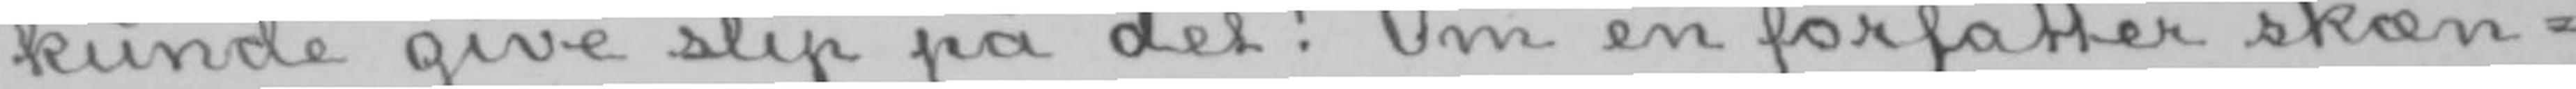kunde give slip på det! Om en forfatter skæn-
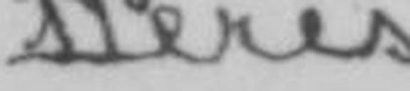Deres
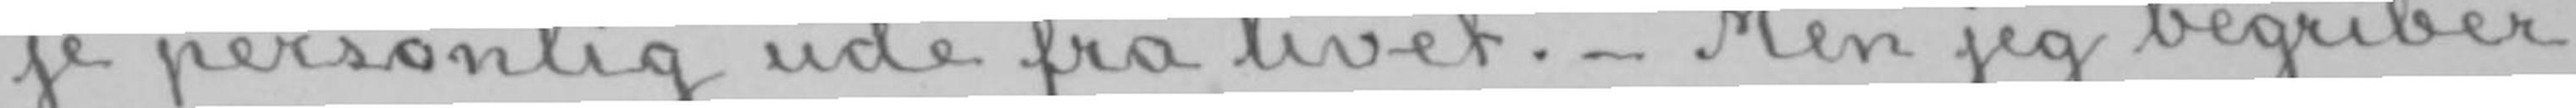je personlig ude fra livet.- Men jeg begriber
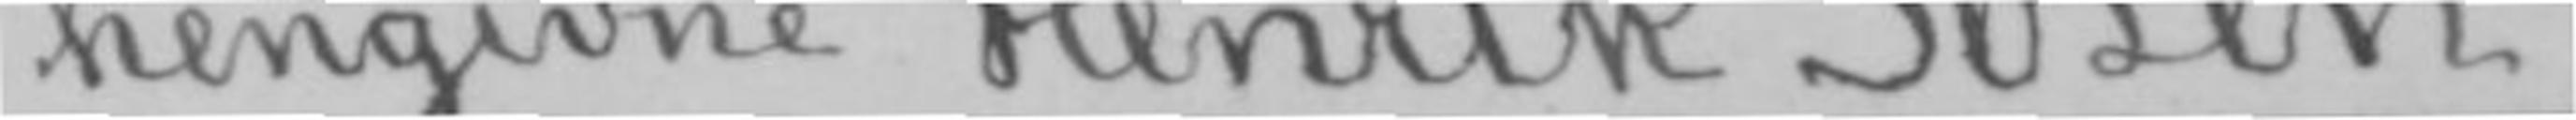hengivne Henrik Ibsen.
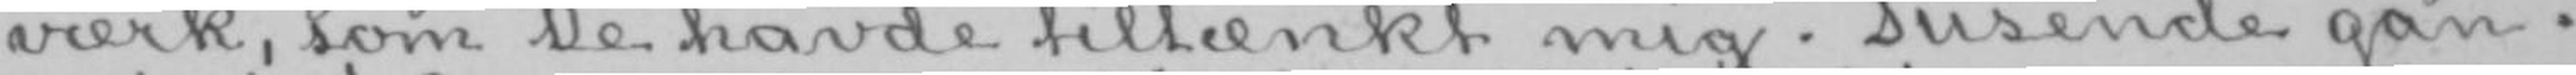værk, som De havde tiltænkt mig. Tusende gan-
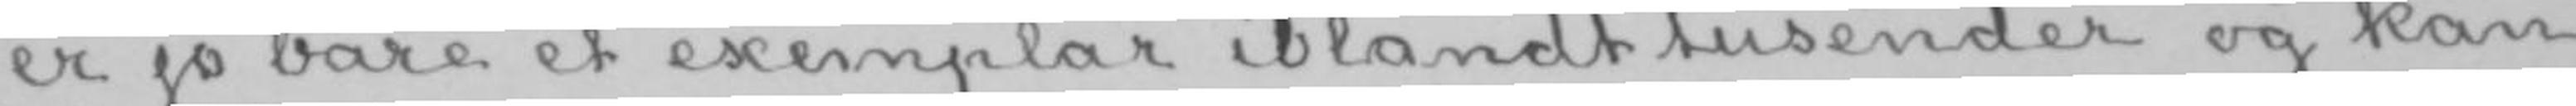er jo bare et exemplar iblandt tusender og kan
